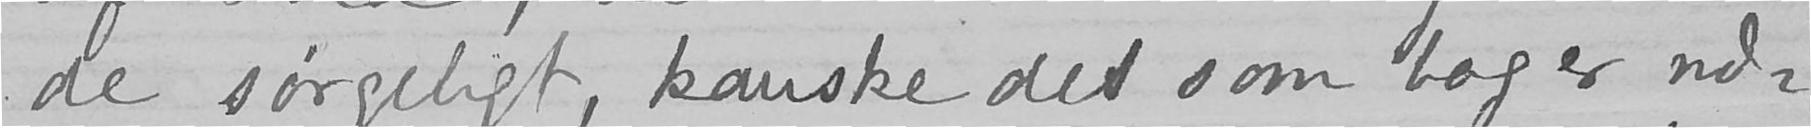de sørgeligt, kanske det som bog er næ-
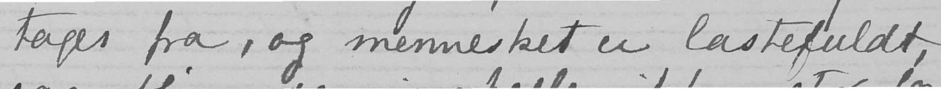tages fra, og mennesket er lastefuldt,
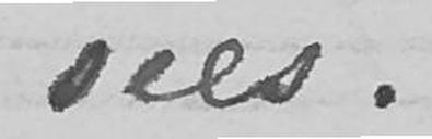sees.
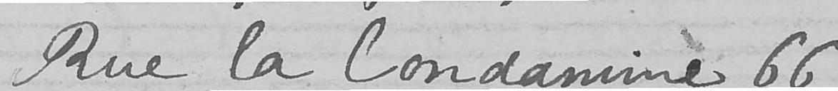Rue la Condamine 66
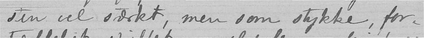sten vel stærkt, men som stykke, for-
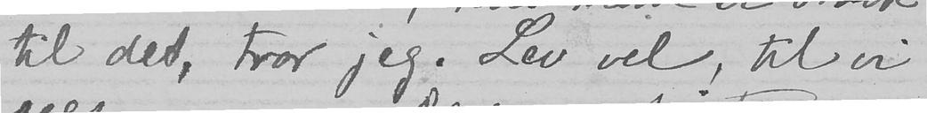til det, tror jeg. Lev vel, til vi
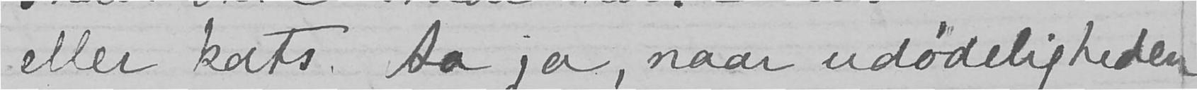eller kats. Ja ja, naar udødeligheden
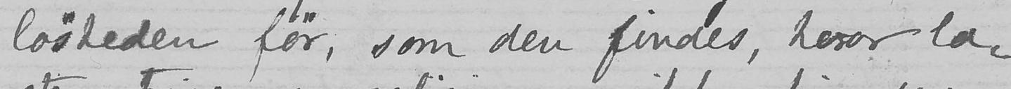løsheden før, som den findes, hvor la-
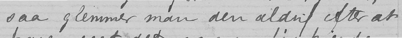saa glemmer man den aldrig efter at
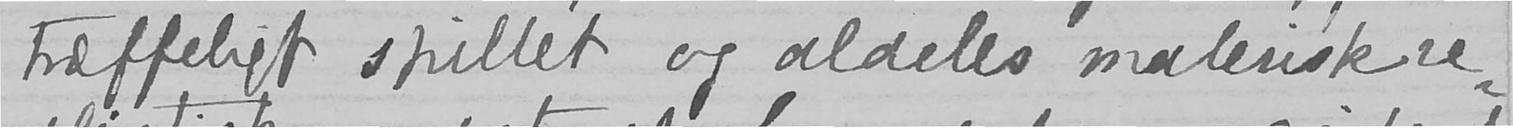træffeligt spillet og aldeles malerisk re-
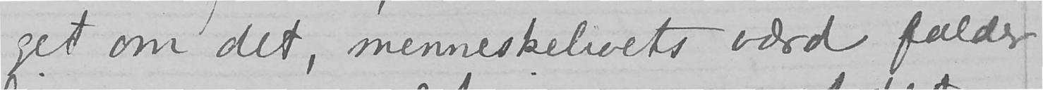get om det, menneskelivets værd falder
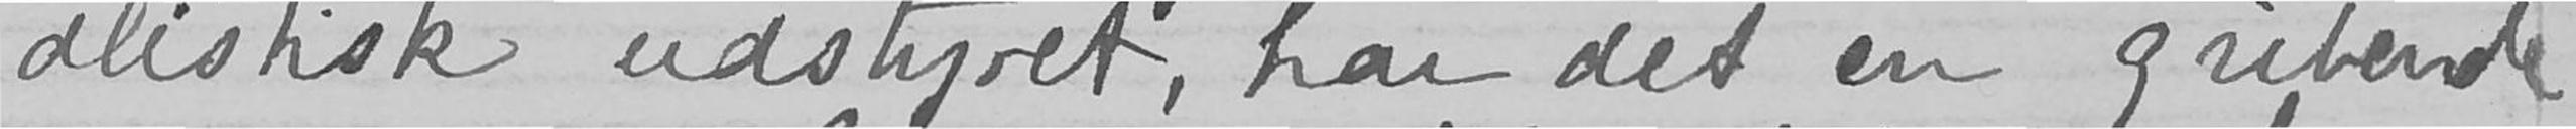alistisk udstyret, har det en gribende
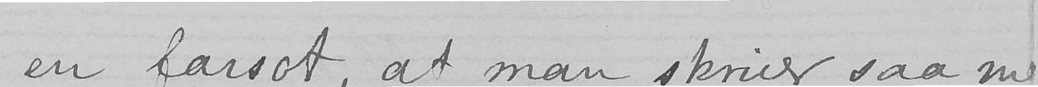en farsot, at man skriver saa me-
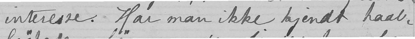interesse. Har man ikke kjendt haab-
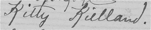Kitty Kielland.
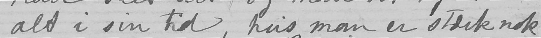alt i sin tid, hvis man er stærk nok
